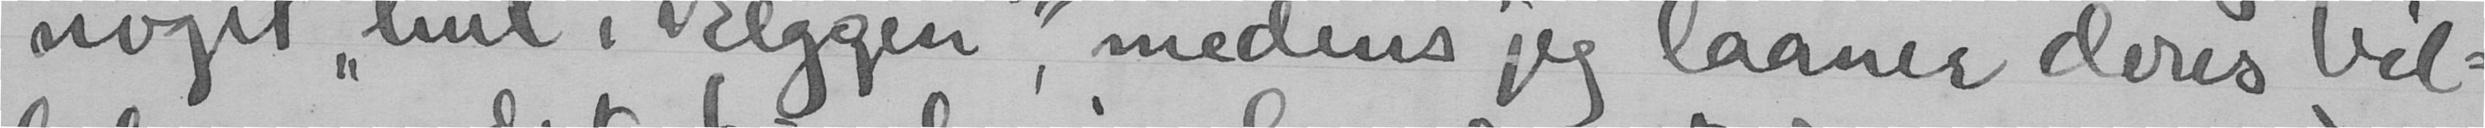noget "hul i væggen", medens jeg laaner deres bil-
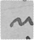med
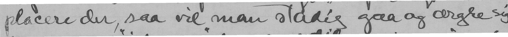placere der, saa vil man stadig gaa og ærgre sig
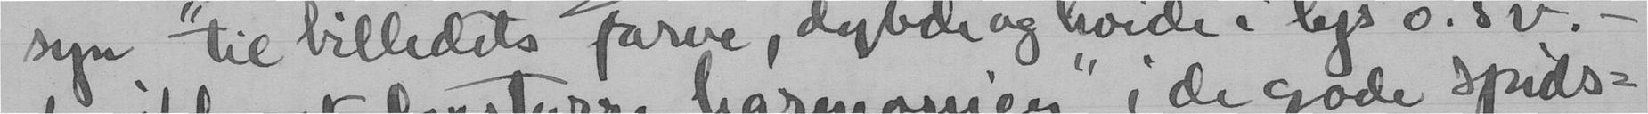syn til billedets farve, dybde og høide i lys o.s.v. -
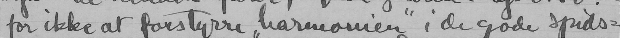for ikke at forstyrre "harmonien" i de gode spuds-
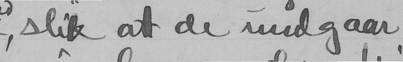, slik at de undgaar
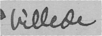billede
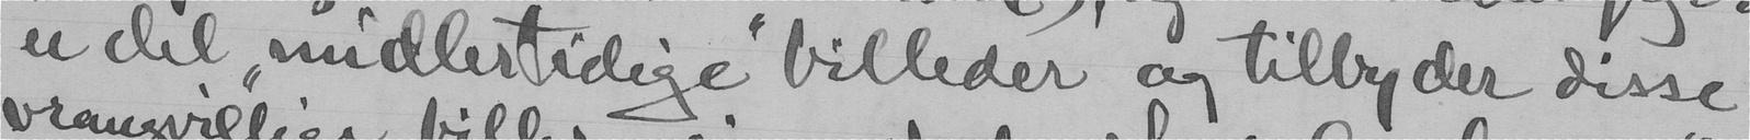er del "midlertidige" billeder og tilbyder disse
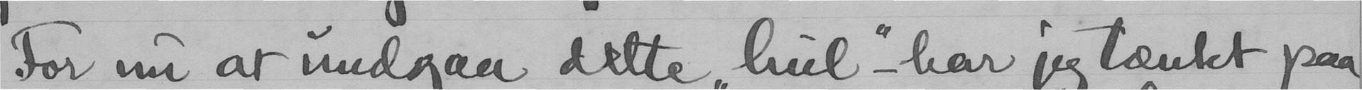For nu at undgaa dette "hul" - har jeg tænkt paa
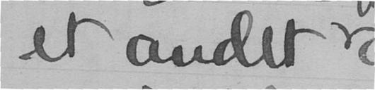et andet
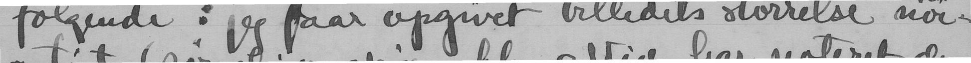følgende : jeg faar opgivet billedets størrelse nøi-
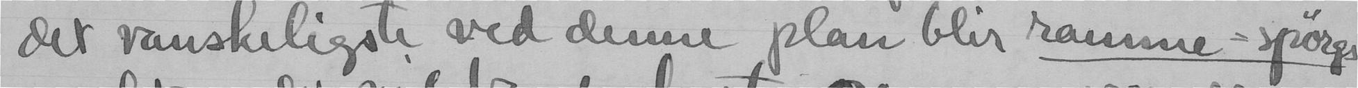det vanskeligste ved denne plan blir ramme -spørgs-
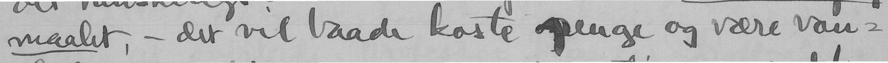maalet - det vel baade koste penge og være van-
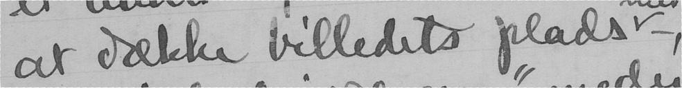at dække billedets plads-
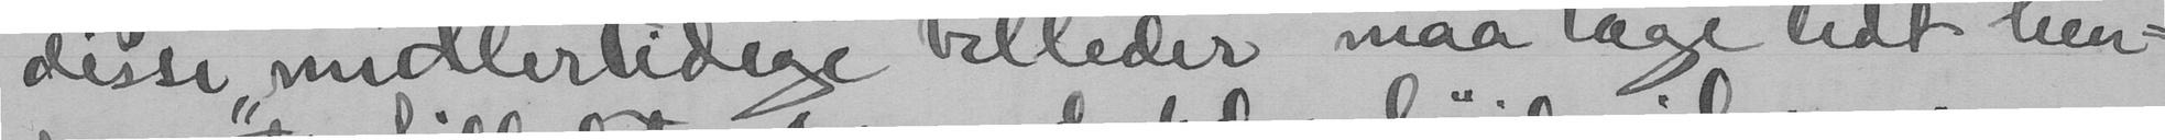disse "midlertidige billeder" maa tage lidt hen-
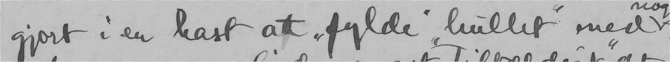gjort i en hast at "fylde" hullet med
